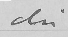din
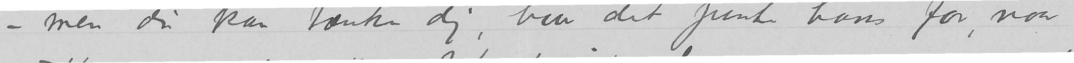men du kan tænke dig, hvor det pinte hans for, naar
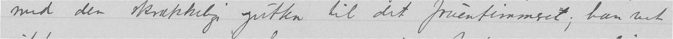med den skrækkelige gutten til det fruentimmeret; han vet
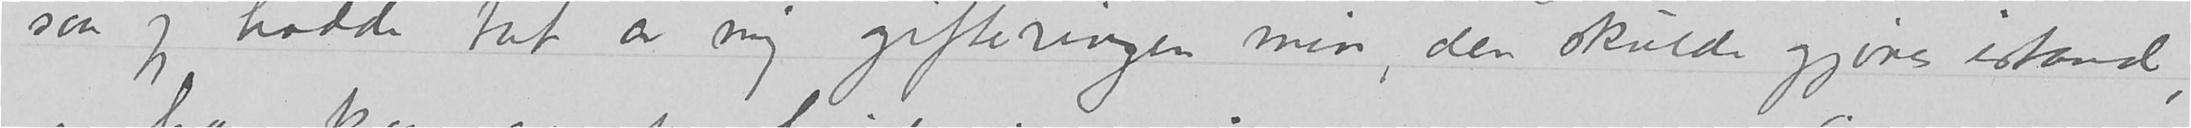saa jeg hadde tat av mig gifteringen min, den skulde gjøre istand
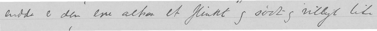endda er den ene altsaa et flinkt og sødt og villigt lite
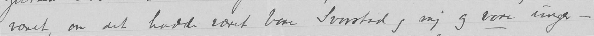været, om det hadde været bare Svarstad og mig og vore unger –
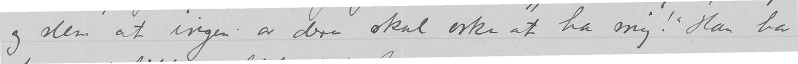og slem at ingen av dere skal orke at ha mig!» Han har
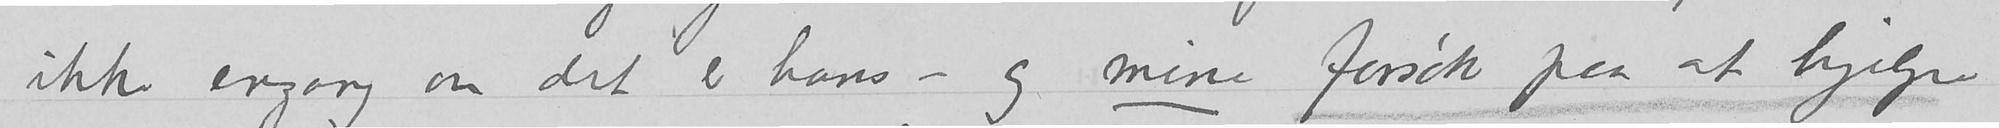ikke engang om det er hans – og mine forsøk paa at hjelpe
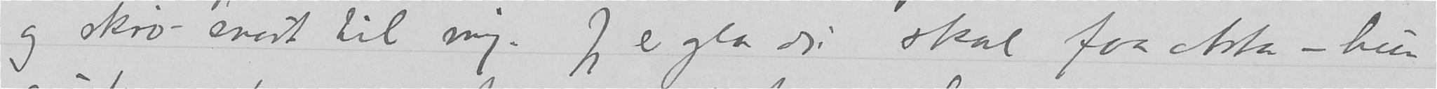og skriv snart til mig. Jeg er gla du skal faa Asta – hun
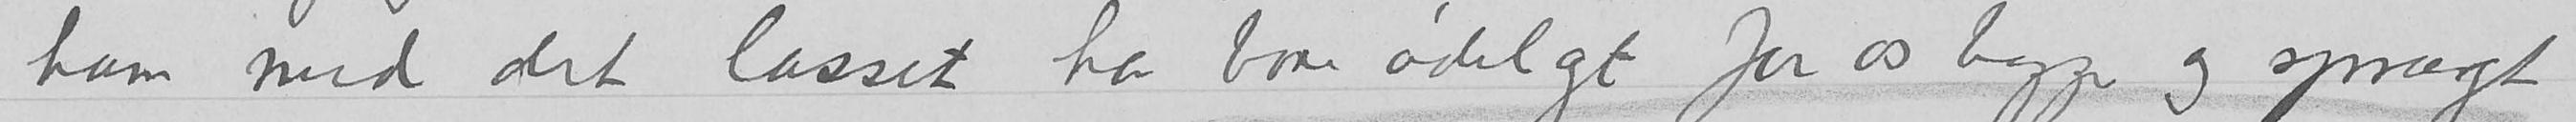ham med det lasset har bare ødelagt for os begge og sprængt
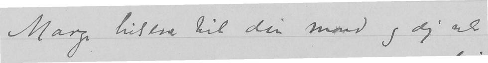Mange hilsener til din mand og dig selv
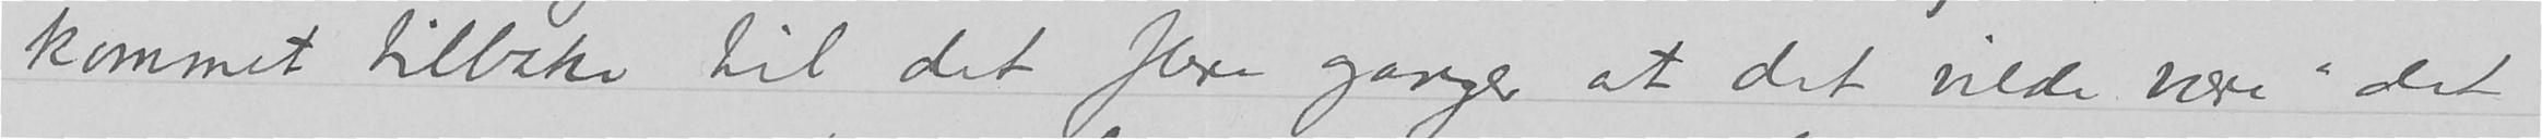kommet tilbake til det flere ganger at det vilde være «det
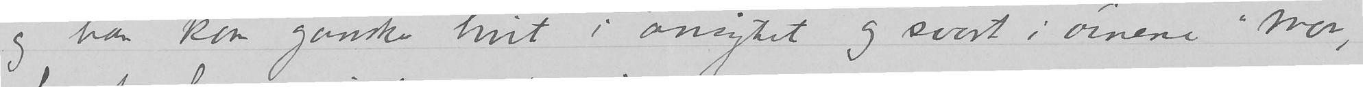og han kom ganske hvit i ansigtet og svart i øinene «mor,
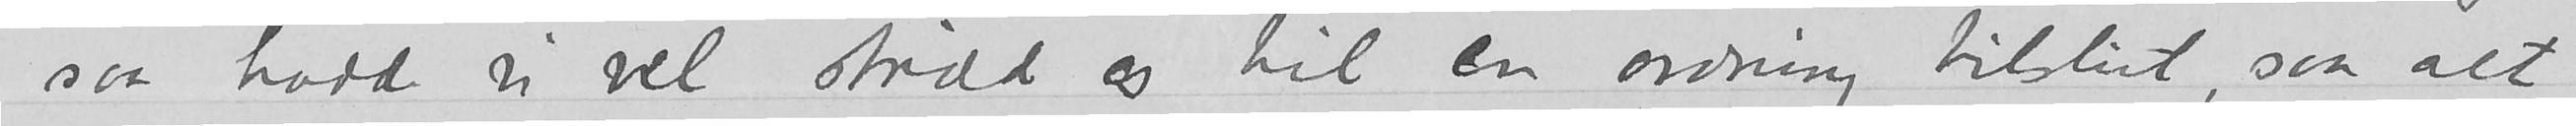saa hadde vi vel stridd og til en ordning tilslut, saa alt
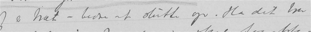Jeg er træt – bedre at slutte op.  Ha det bra
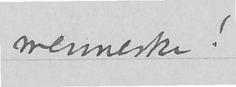menneske!
